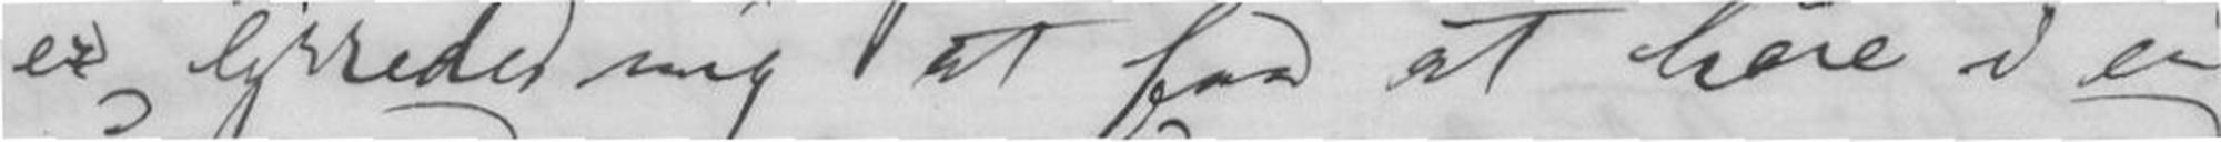er lykkedes mig at faa at høre i en
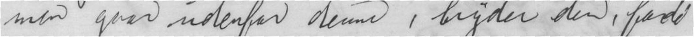men gaar udenfor denne, bryder den, fordi
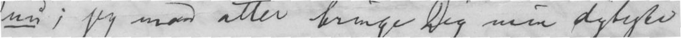nu; jeg maa atter bringe Dig min dybeste
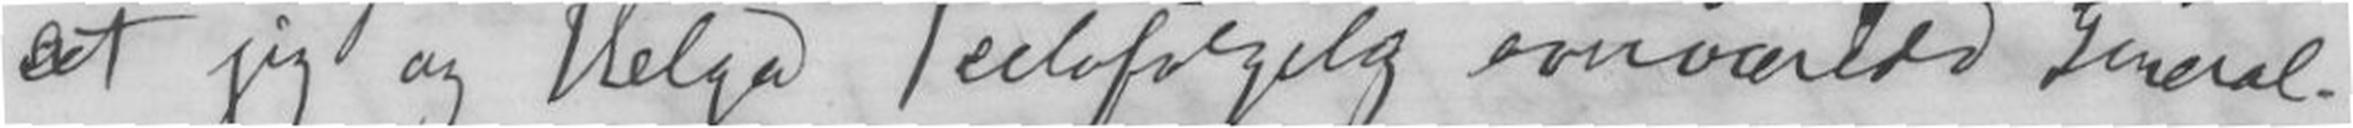at jeg og Helga selvfølgelig overværede General-
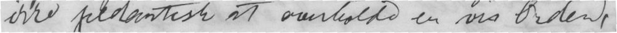ikke pedantisk at overholde en vis Orden,
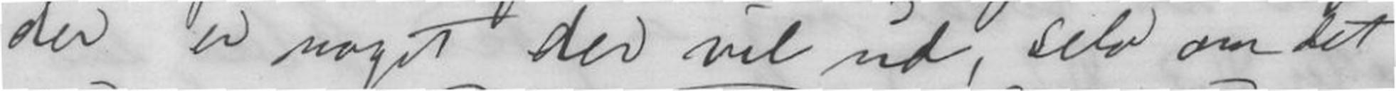der er noget der vil ud, selv om det
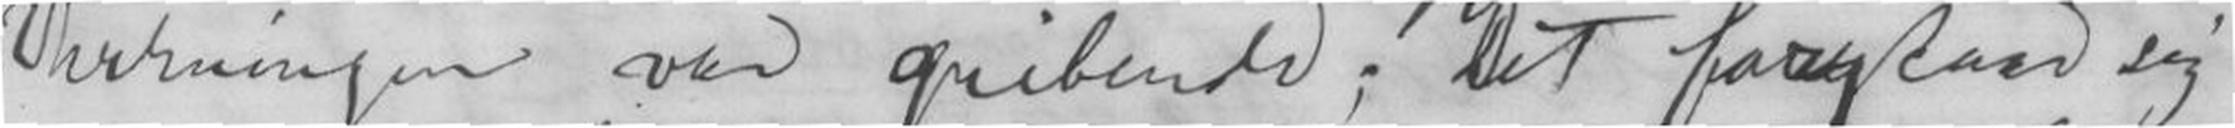Virkningen var gribende; Det forstaar sig
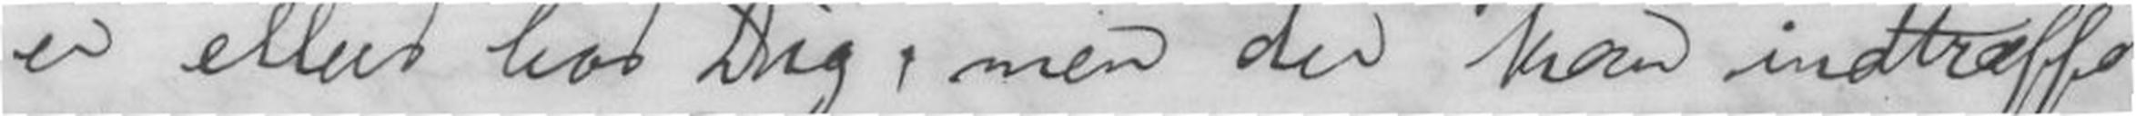er ellers hos Dig, men der kan indtræffe
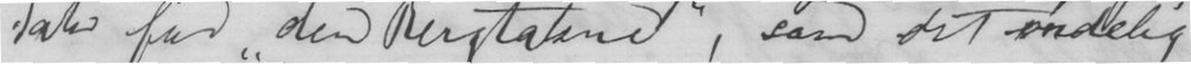Tak for den Bergtekne, som det endelig
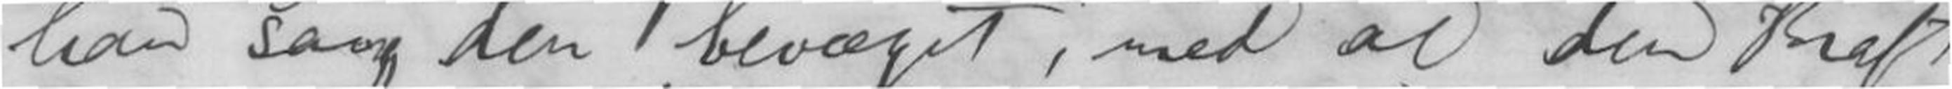han sang den bevæget, med al den Kraft
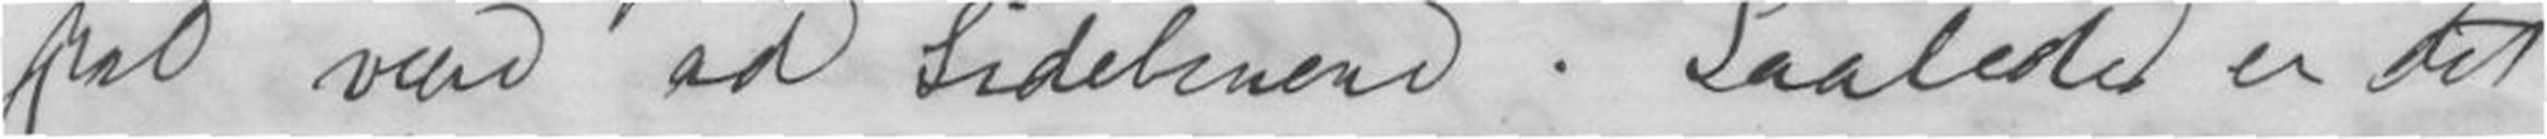skal være ad Sidebenene. Saaledes er det
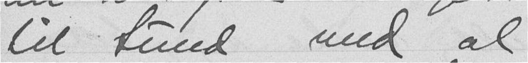til Sund med al
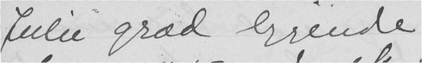Julie græd liggende
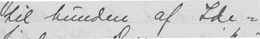til bunden af Ide-
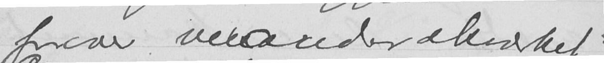forover verandarækværket,
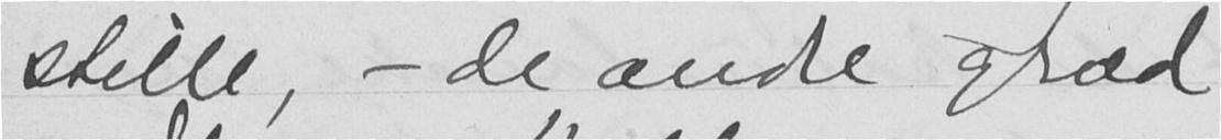stille, - de andre græd
